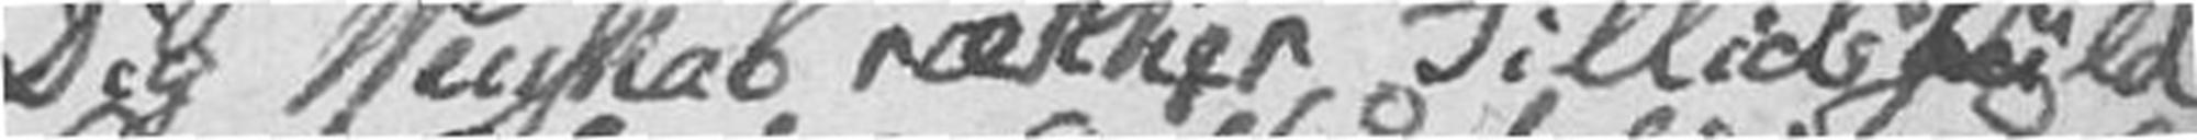Dig Venskab rækker Tillidsfuld
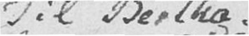Til Bertha!
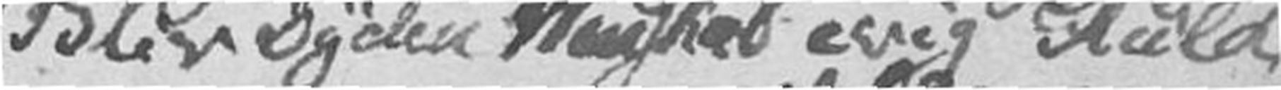Bliv Dyden Venskab evig Huld
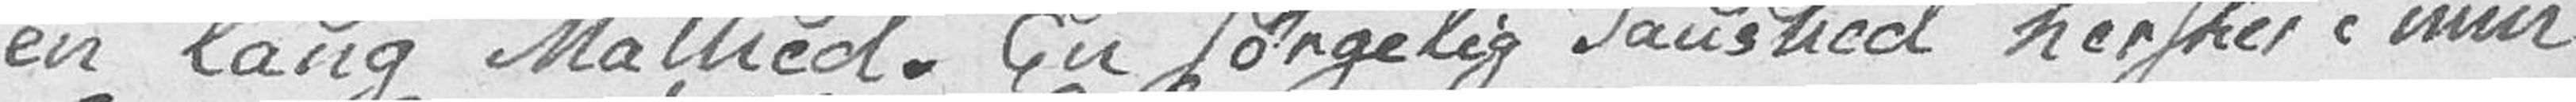en lang Mathed. En sørgelig Taushed hersker i min
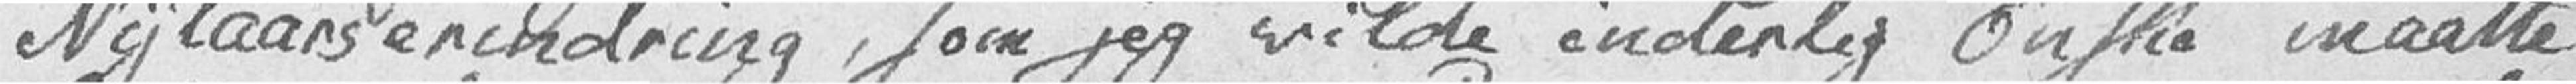Nytaarserindring, som jeg vilde inderlig Ønske maatte
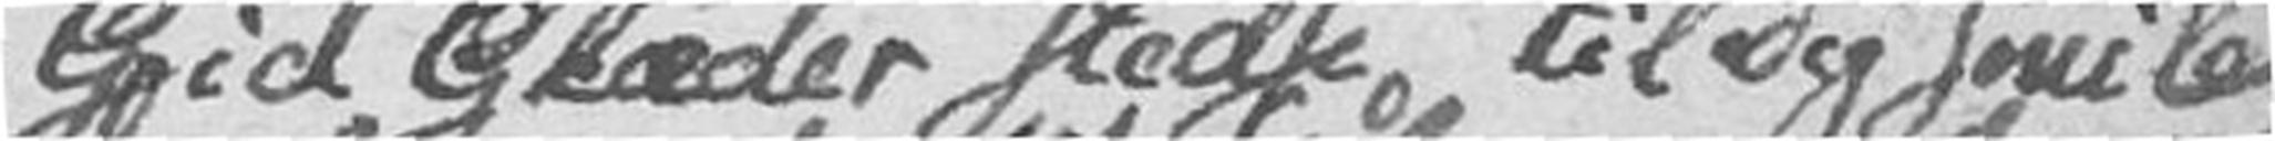Gid Glæder stedse til dig smile
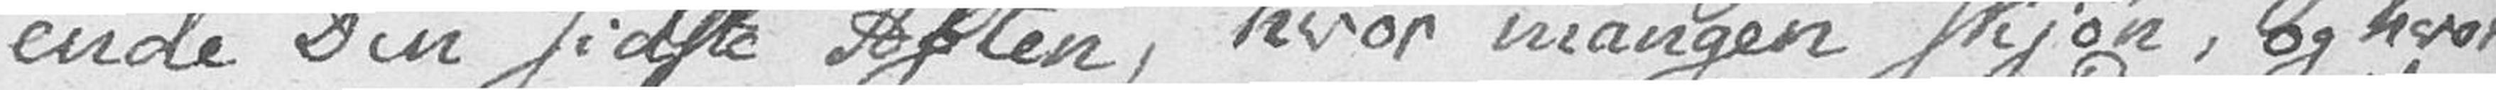ende Din sidste Aften, hvor mangen skjøn, og hvor
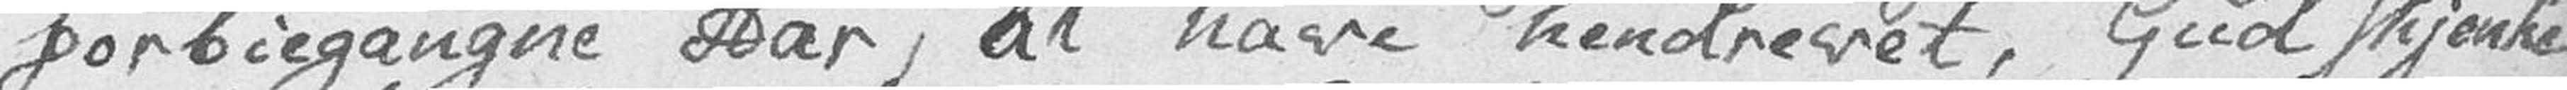forbiegangne Aar, at have hendrevet, Gud skjenke
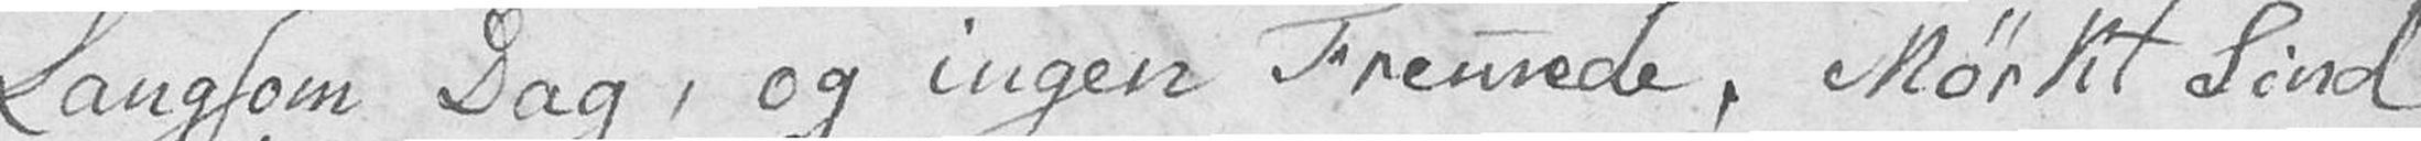Langsom Dag, og ingen Fremmede, Mørkt Sind
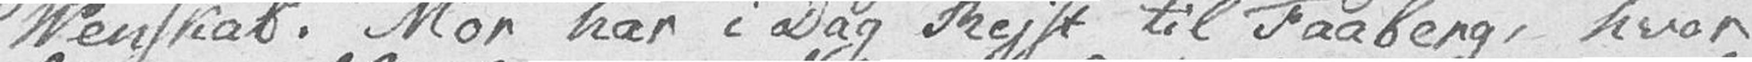Venskab. Mor har i Dag Rejst til Faaberg, hvor
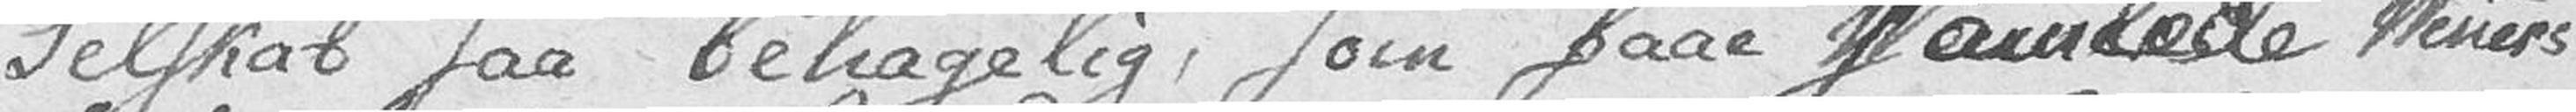Selskab saa behagelig, som faae Samlede Venners
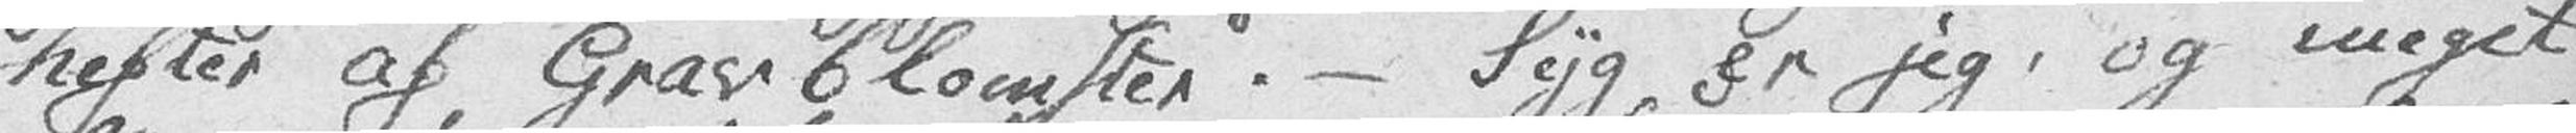hefter af Gravblomster. – Syg er jeg, og meget
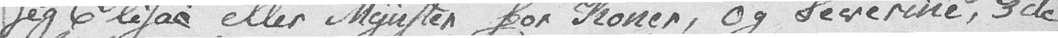jeg Elisae eller Mynster for Koner, og Severine, 3de
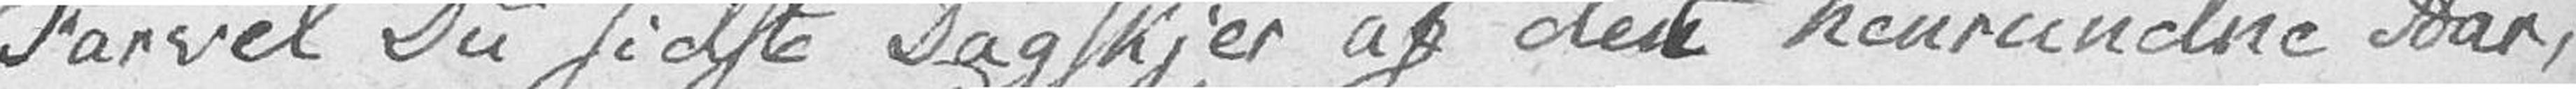Farvel Du sidste Dagskjer af det henrundne Aar,
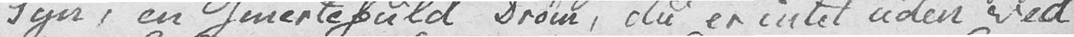Syn, en Smertefuld Drøm, du er intet uden ved
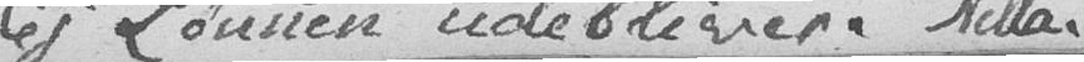Ej Lønnen udebliver. Nella.
